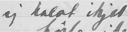sig halvt ihjel
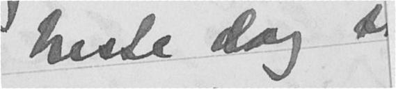neste dag s
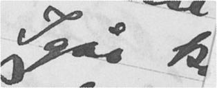Igår k
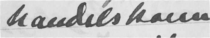handelskance
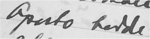Opsto hadde
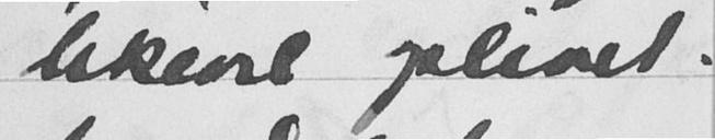likevel oplivet.
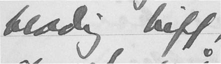blodig biff
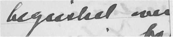begeistret over
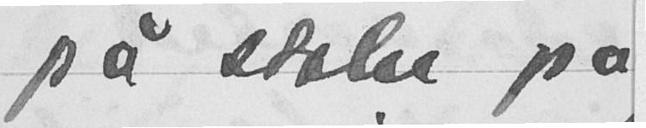på stolen pa
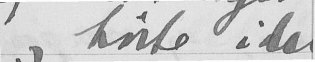og hørte idag
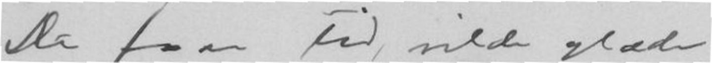De faar Tid, vilde glæde
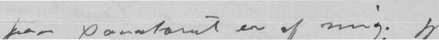paa Sanatoriet er af mig; jeg
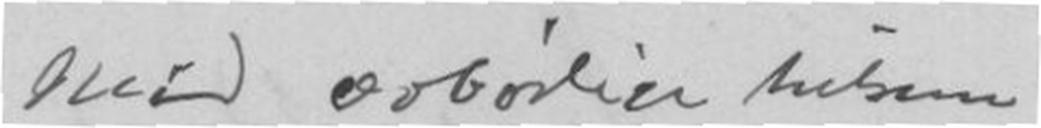Skal overbringe hilsen
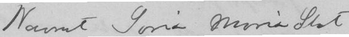Navnet Soria Moria Slot
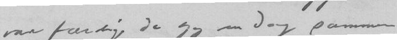var færdig, da jeg en dag sammen
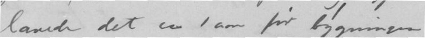lavede det ca 1 aar før bygningen
